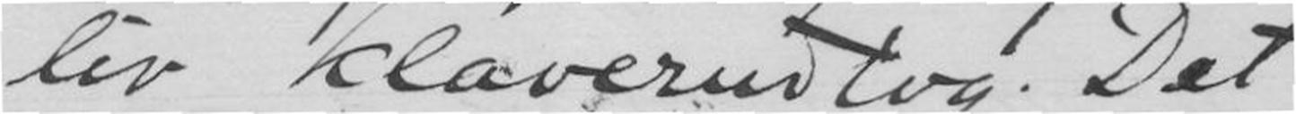liv klaverudtog. Det
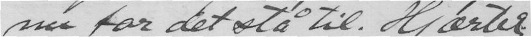nu for det stå til. Hjærtelig
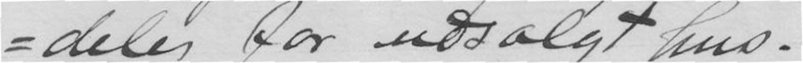deles for udsolgt hus.
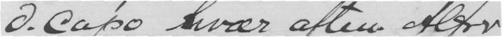d.Capo hvær aften. Alfr
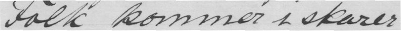Folk kommer i skarer
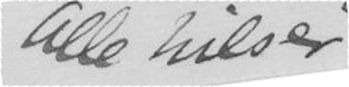Alle hilser
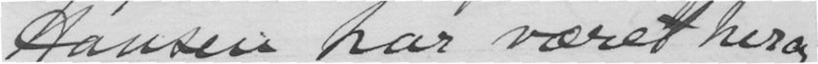Hansen har været her og
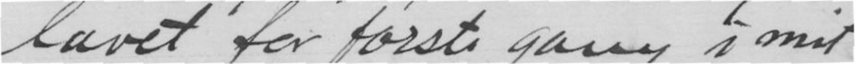lavet for første gang i mit
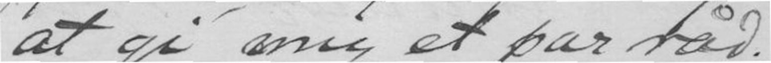at gi mig et par råd. Ja
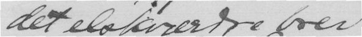det elskværdige brev.
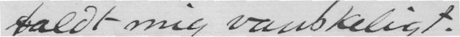faldt mig vanskeligt.
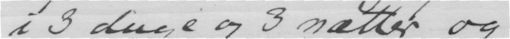i 3 dage og 3 nætter og
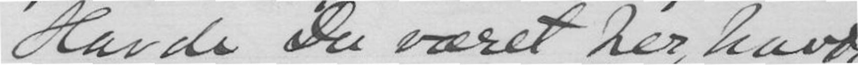Havde Du været her havde
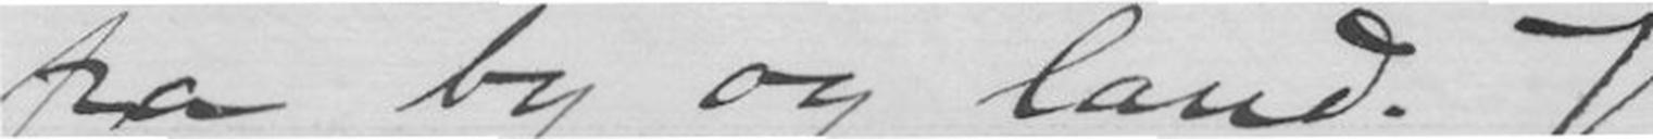fra by og land. Jeg
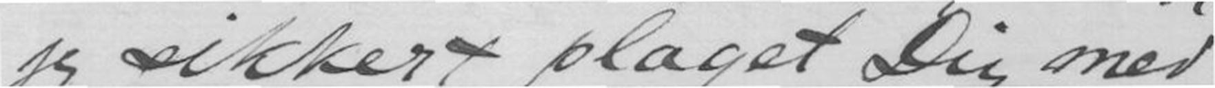jeg sikkert plaget Dig med
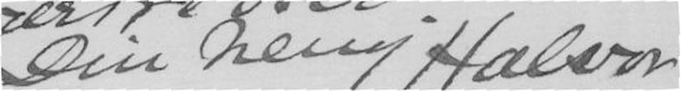Din heng. Halvor
